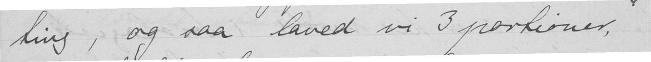ting, og saa laved vi 3 portioner,
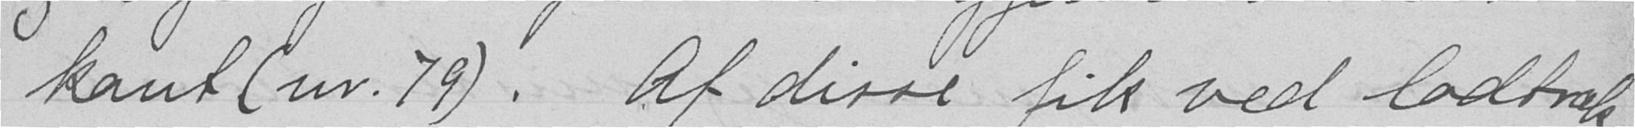kant (nr. 79). Af disse fik ved lodtræk-
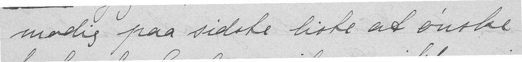modig paa sidste liste at ønske
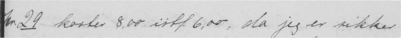Nr. 29 koster 8,00 istf. 6,00, da jeg er sikker
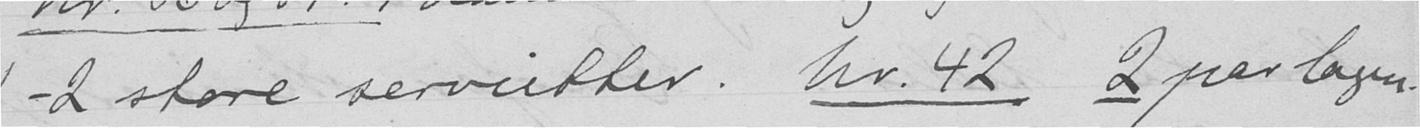1-2 store servietter. Nr. 42 2 par lagen.
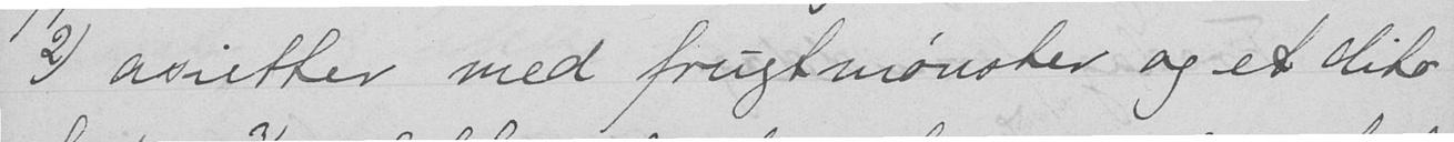2) asietter med frugtmønster og et lite
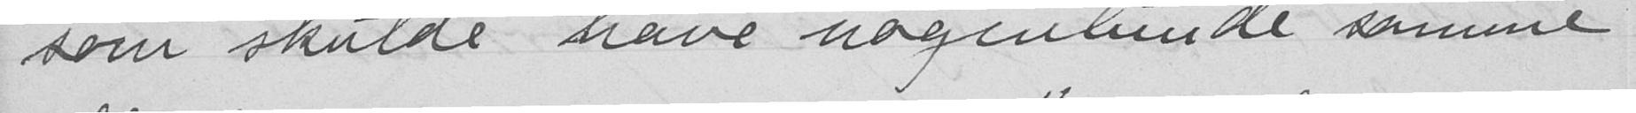som skulde have nogenlunde samme
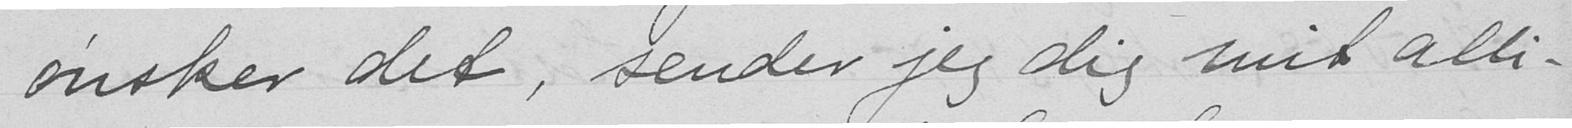ønsker det, sender jeg dig mit alli-
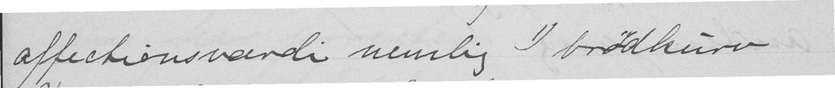affectionsværdi nemlig 1) brødkurv
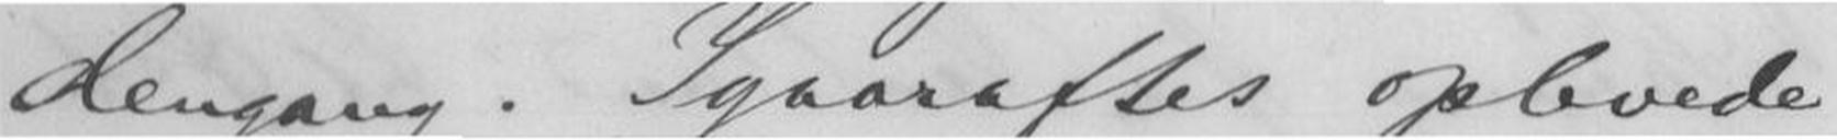dengang. Igaaraftes oplevede
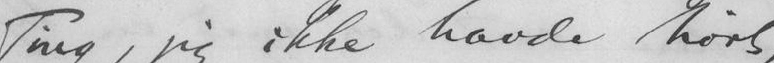Ting, jeg ikke havde hørt,
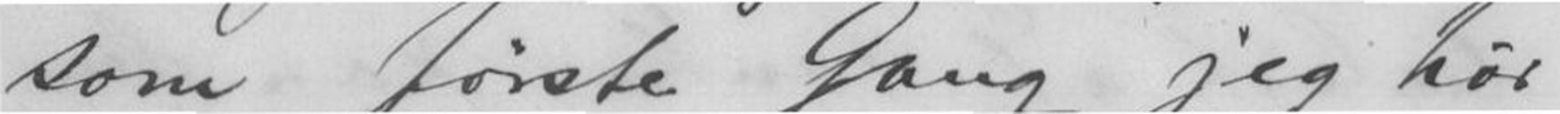som første Gang jeg hør-
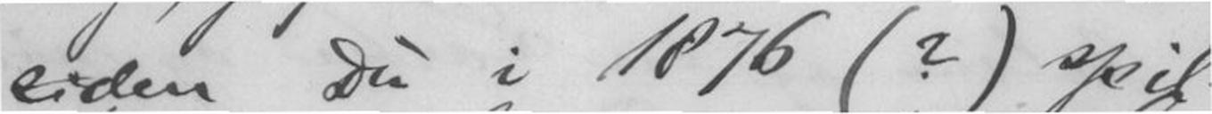siden Du i 1876 (?) spil-
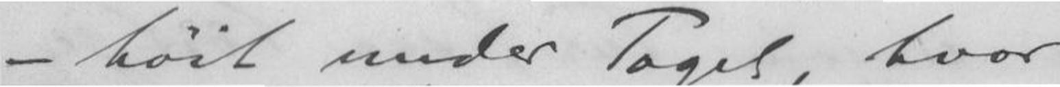- høit under Taget, hvor
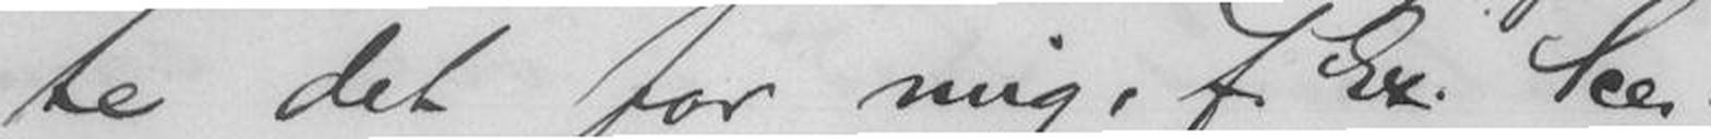te det for mig, f Ex. Sce-
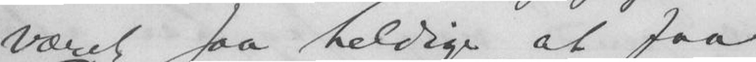været saa heldige at faa
